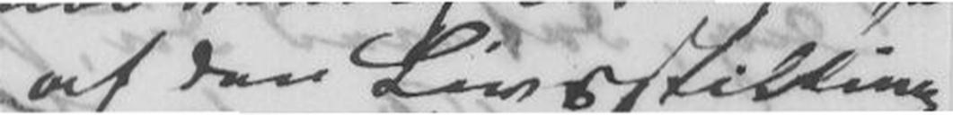af den Livsstilling
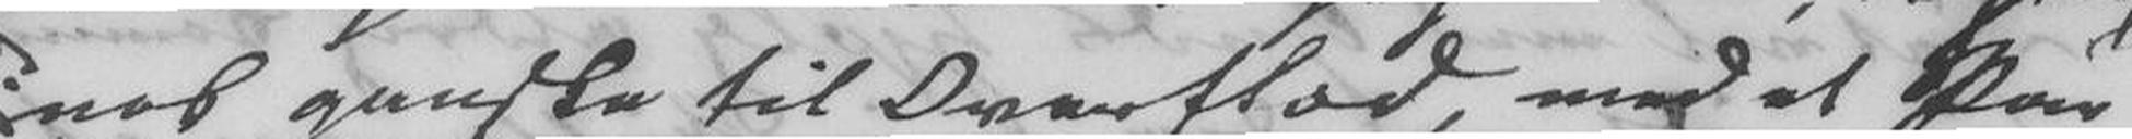nok ganske til Overflod, med et Par
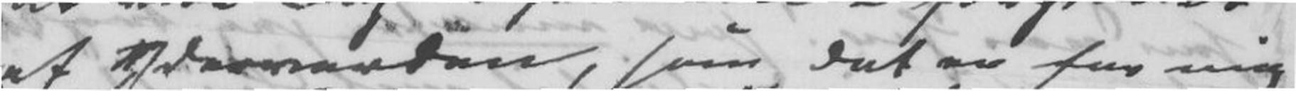af Ydermaaden, som det er for mig
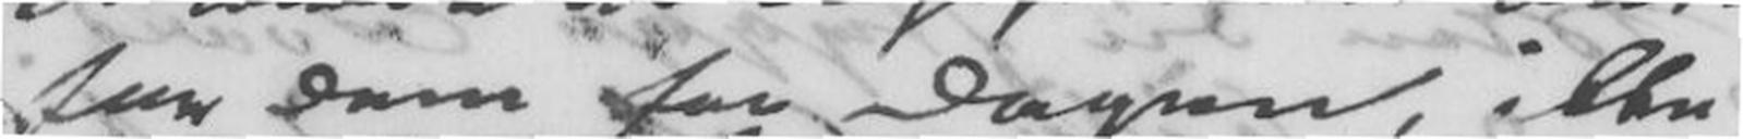for Dem for Dagen, ikke
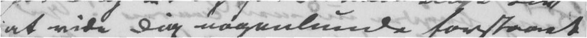at vide Dig nogenlunde forstaaet
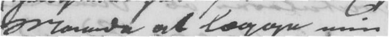Mande at lægge min
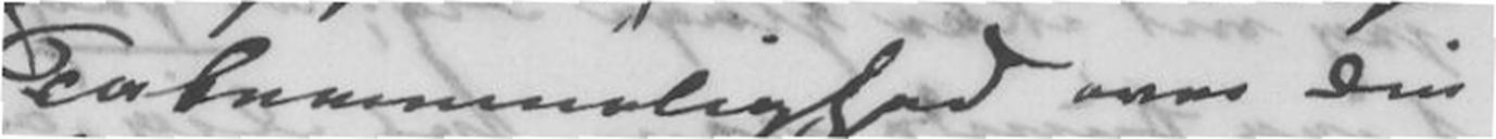Taknemmelighed over Din
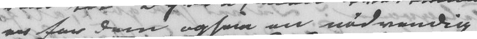er for dem ogsaa en nødvendig
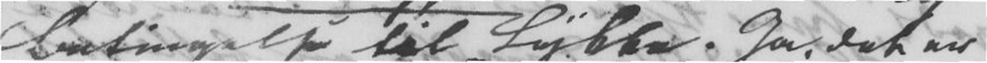betingelse til Lykke. Ja, det er
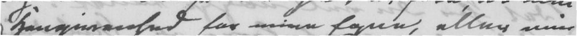hængivenhed for mine Egne, eller min
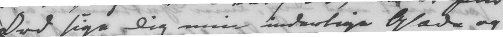Ord sige Dig min inderlige Glæde og
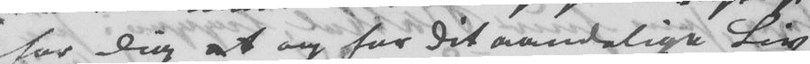for Dig og for Dit aandelige Liv
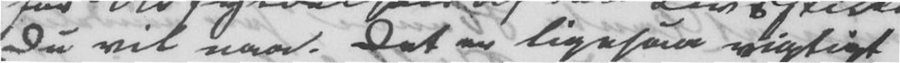Du vil naa. Det er ligesaa vigtigt
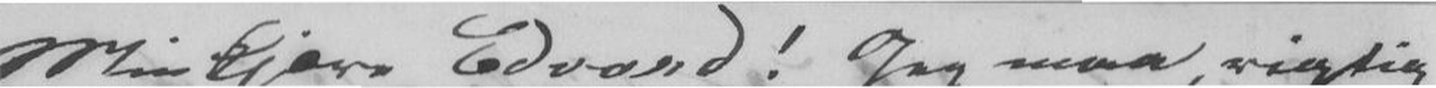Min kjære Edvard! jeg maa, rigtig
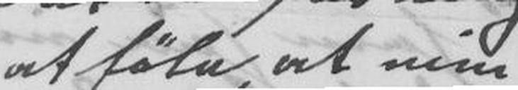at føle, at min
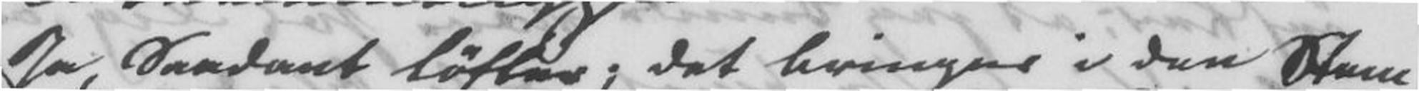Ja, Saadant løfter; det bringer i den Stem
